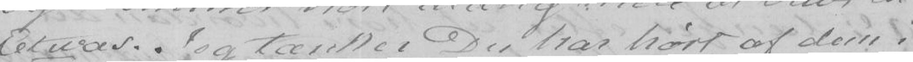Etwas. Jeg tænker Du har hørt af dem i
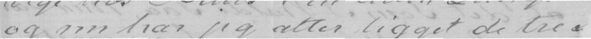og nu har jeg atter ligget de tre
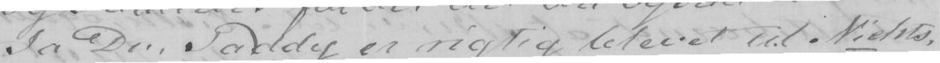Ja Din Paddy er rigtig blevet til Nichts,
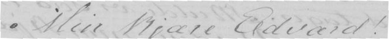Min kjære Edvard!
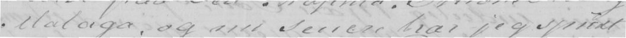Malaga, og nu senere har jeg spiist
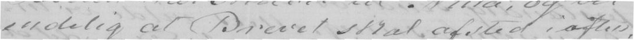endelig at Brevet skal afsted iaften,
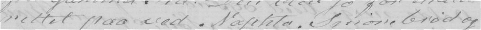rettet paa ved Naptha. Smørebrød og
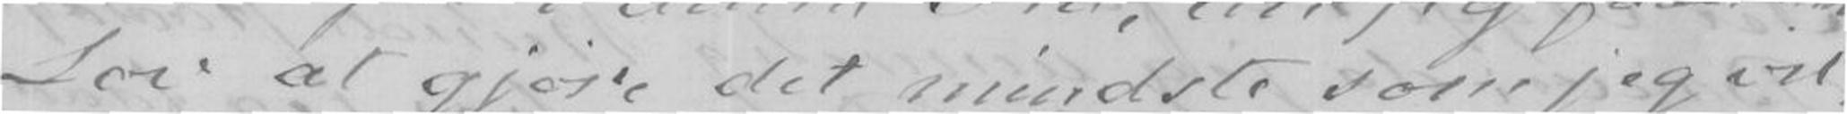Lov at gjøre det mindste som jeg vil,
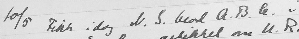10/5 Fikk. i dag N. S. blad A.B.C. i
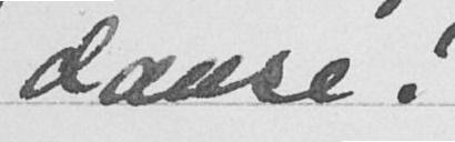danse.
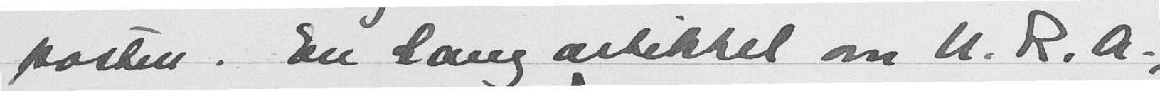posten. En lang artikkel om N. R. A.
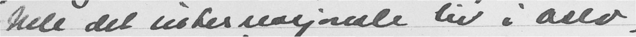hele det internasjonale liv i selv,
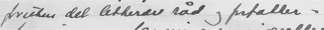foruten det litterære råd og forfatter-
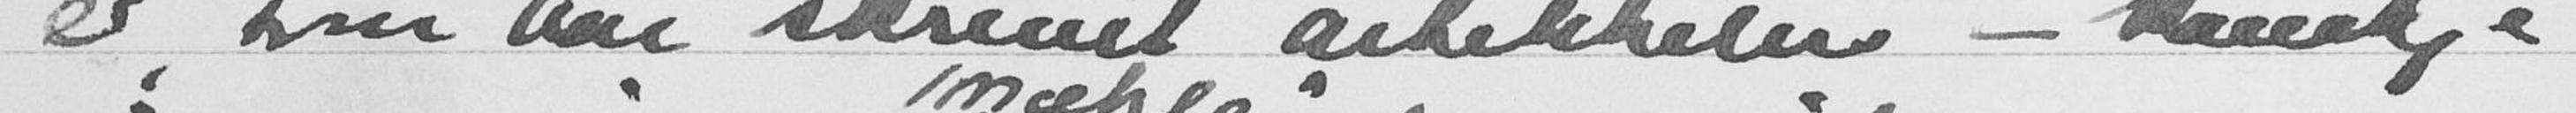er, som har skrevet artikkelen - kanskje
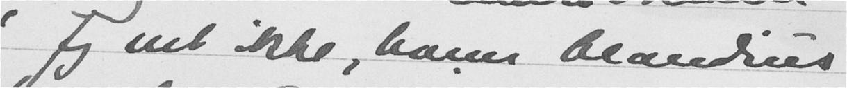Jeg vet ikke, hvem klandrius
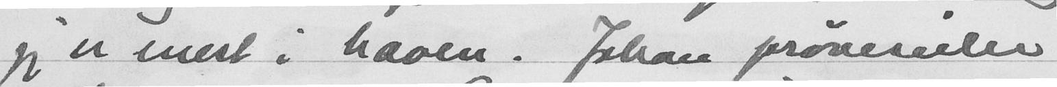jeg er mest i haven. Johan prøveseiler
